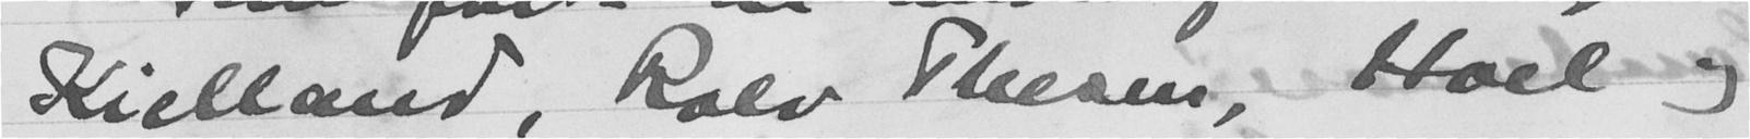Kielland, Rolv Thesen, Hoel og
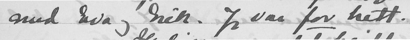med Eva og Erik. Jeg var for trøtt.
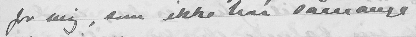for mig, som ikke har såmange
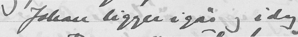Johan ligger igår og i dag
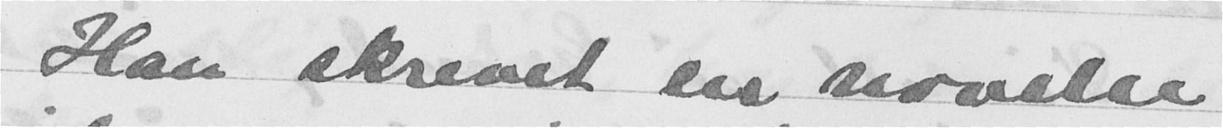Har skrevet en novelle
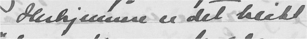Herjemme er det blitt
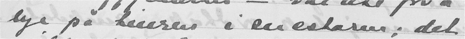lye på venren i en estarm; det
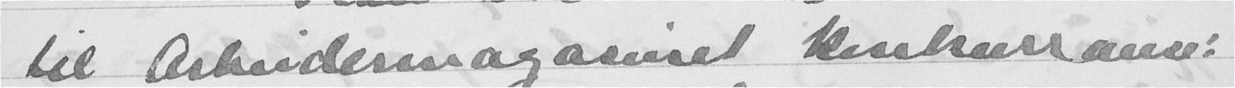til Arbeidermagasinet konkurranse:
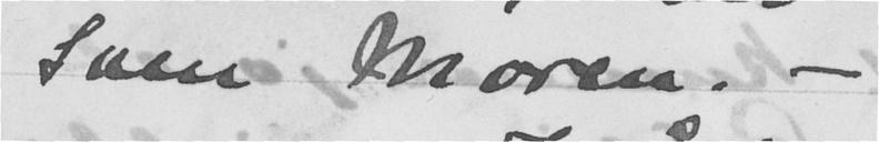Som Moren. -
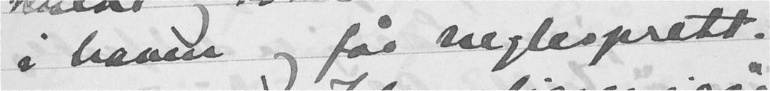i haven og får neglesprett.
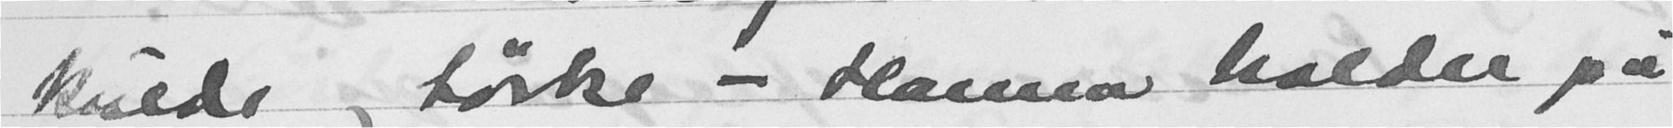kulde, tørke - Hanna holder på
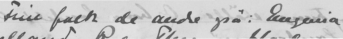Fine folk de andre også: Eugenia
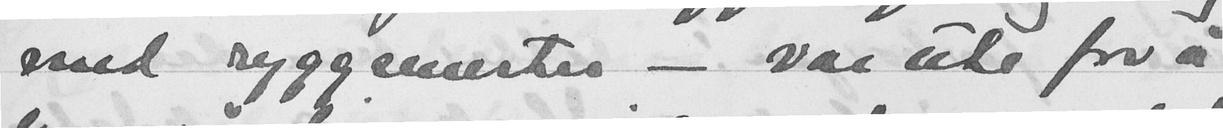med ryggsmertet - var ute for å
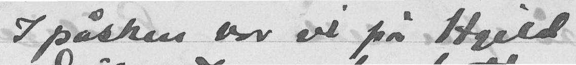I påsken var vi på Hgild
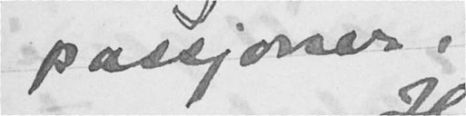passjoner.
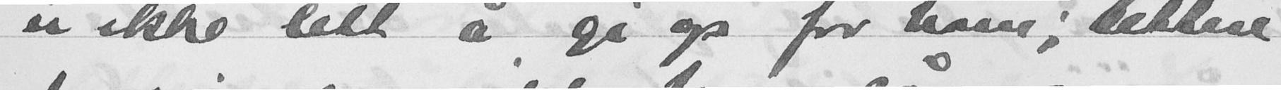er ikke lett i gi op for ham; lettere
